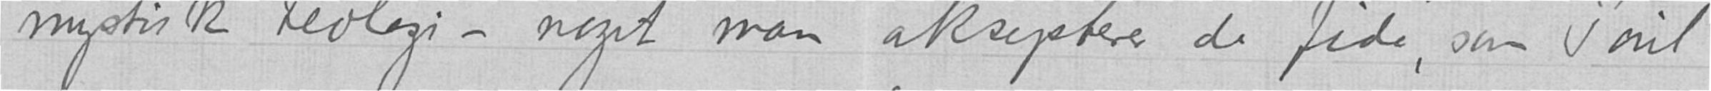mystisk teologi – noget man aksepterer de fide, som Paul
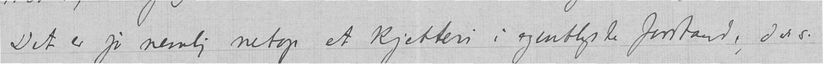Det er jo nemlig netop et kjetteri i egentligste forstand, dvs.
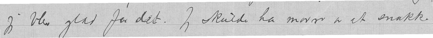jeg blev glad for det. Jeg skulde ha morro av at snakke
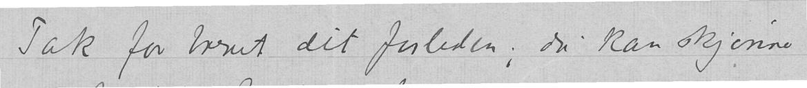Tak for brevet dit forleden; du kan skjønne
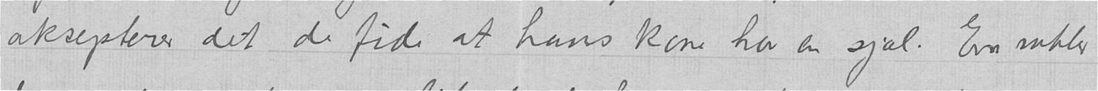aksepterer det de fide at hans kone har en sjæl. En vakker
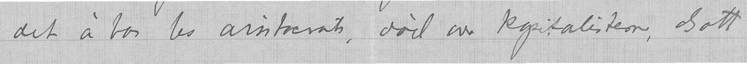det à bas les aristocrats, død over kapitalisterne, Gott
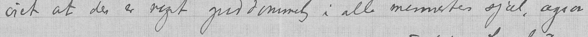øiet at der er noget guddommelig i alle menneskers sjæl, ogsaa
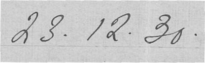23.12.30.
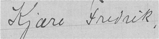Kjære Fredrik,
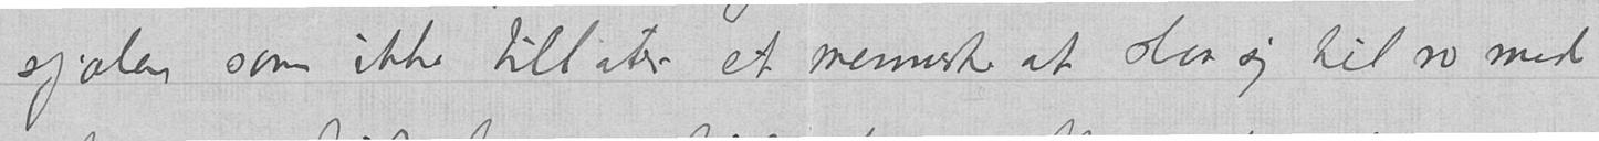sjælen som ikke tillater et menneske at slaa sig til ro med
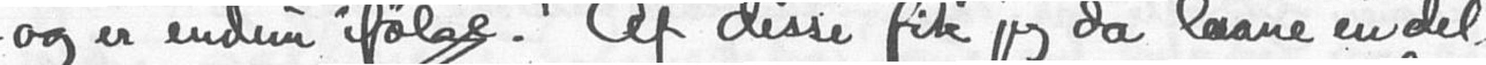- og er endnu ifølge. Af disse fik jeg da laane en del
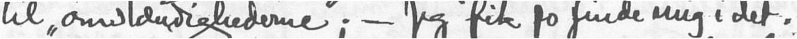til "omstændighederne". - Jeg fik jo finde mig i det.
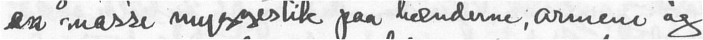en masse myggestik paa hænderne, armene og
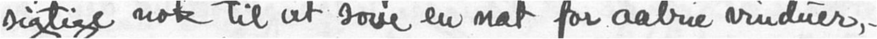sigtige nok til at sove en nat for aabne vinduer, -
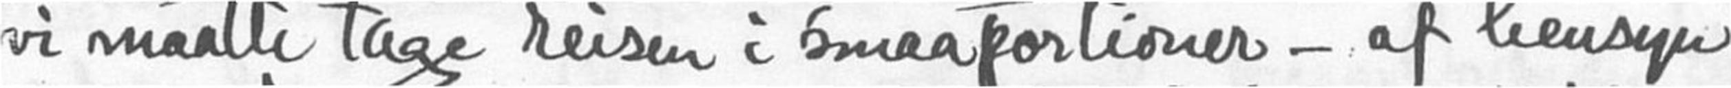vi maatte tage reisen i smaaportioner - af hensyn
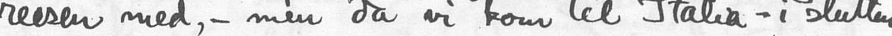reisen med, - men da vi kom til Italia - i slutten
